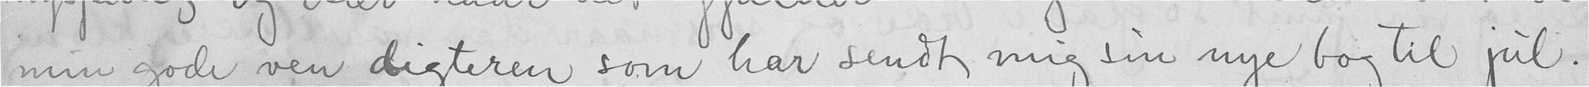min gode ven digteren som har sendt mig sin nye bog til jul.
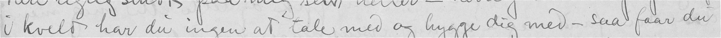i kveld har du ingen at tale med og hygge dig med, - saa faar du
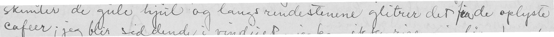skimter de gule hjul og langs rundestenene glitrer det finde oplyste
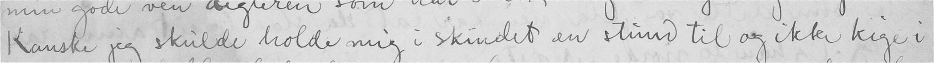Kanske jeg skulde holde mig i skindet en stund til og ikke kige i
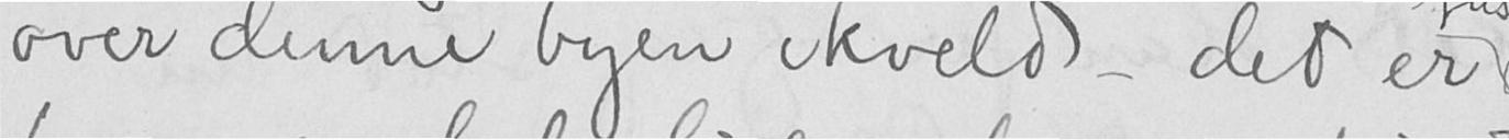over denne byen ikveld - det er
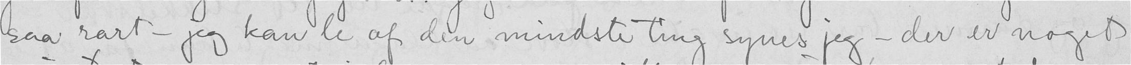saa rart - jeg kan de af den mindste ting synes jeg - der er noget
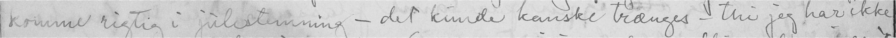komme rigtig i julestemning, - det kunde kanske trænges, thi og har ikke
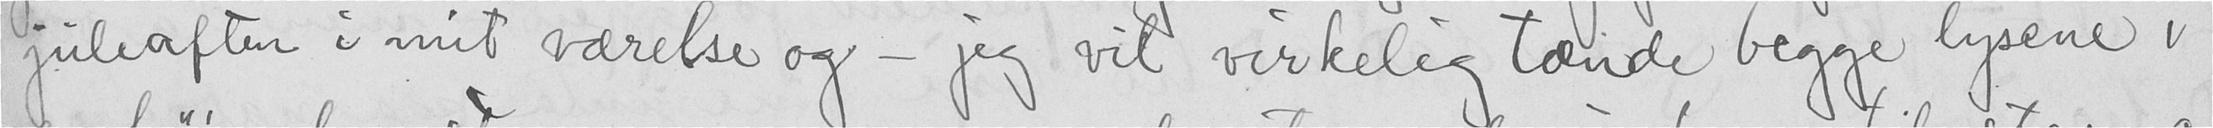juleaften i mit værelse og - jeg vil virkelig tænde begge lysene i
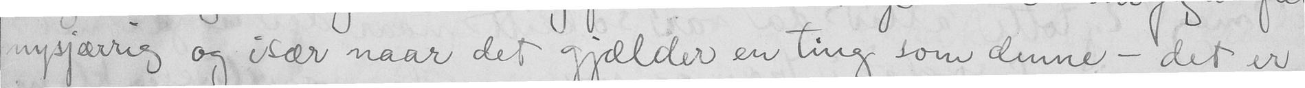nysjærrig og især naar det gjælder en ting som denne - det er
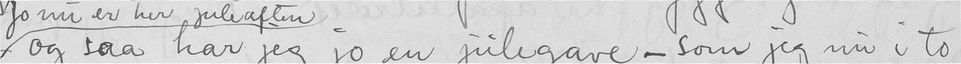og saa har jeg jo en julegave, - som jeg nu i to
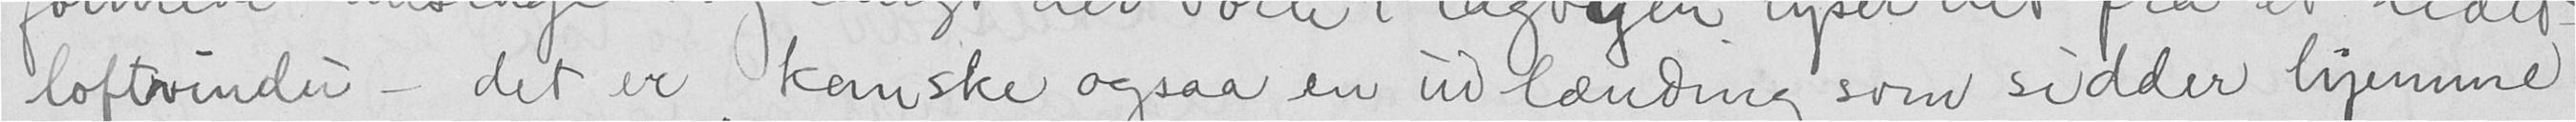loftvindu - det er kanske ogsaa en udlænding som sidder hjemme
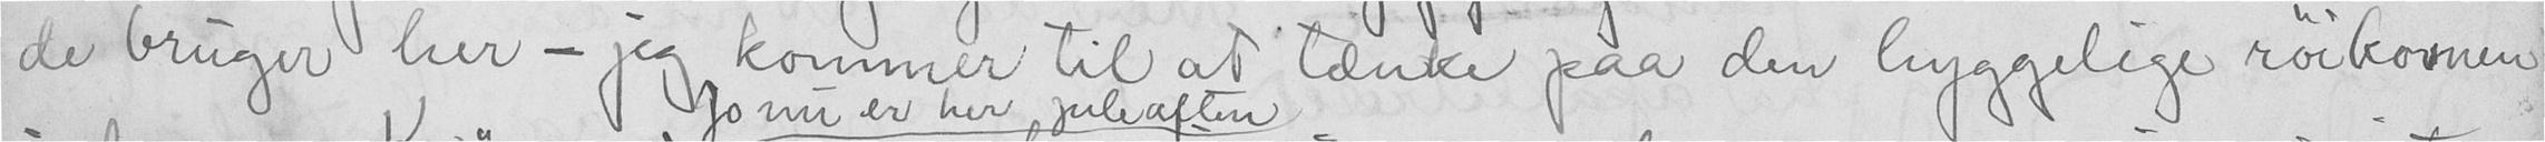de bruger her - jeg kommer til at tænke paa den hyggelige røikovnen
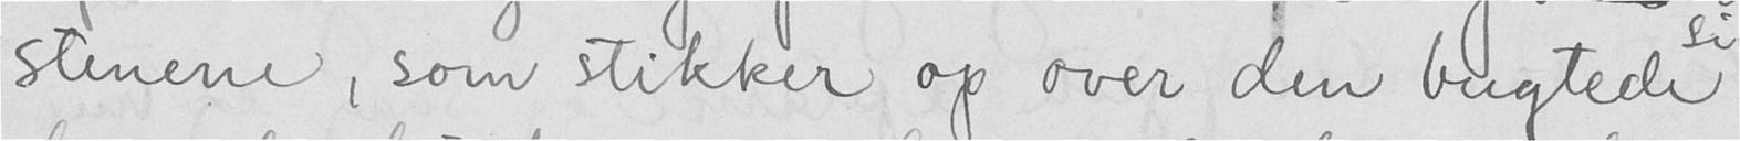stenene, som stikker op over den bugtede
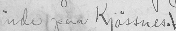inde paa Kjøssnes
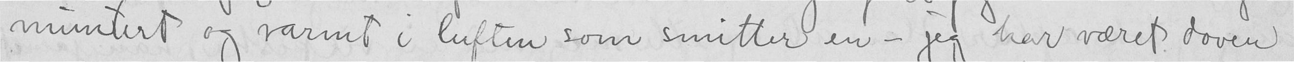muntert og varmt i luften som smitter en - jeg har været doven
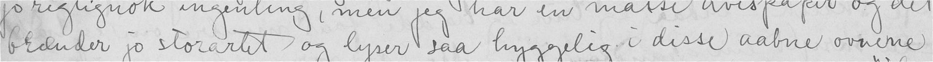brænder jo storartet og lyser saa hyggelig i disse aabne overne
